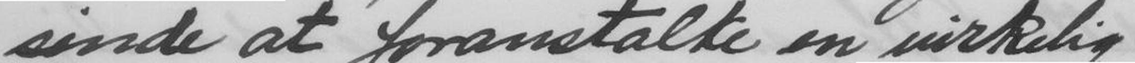sinde at foranstalte en virkelig
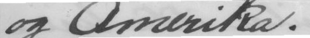og Amerika.
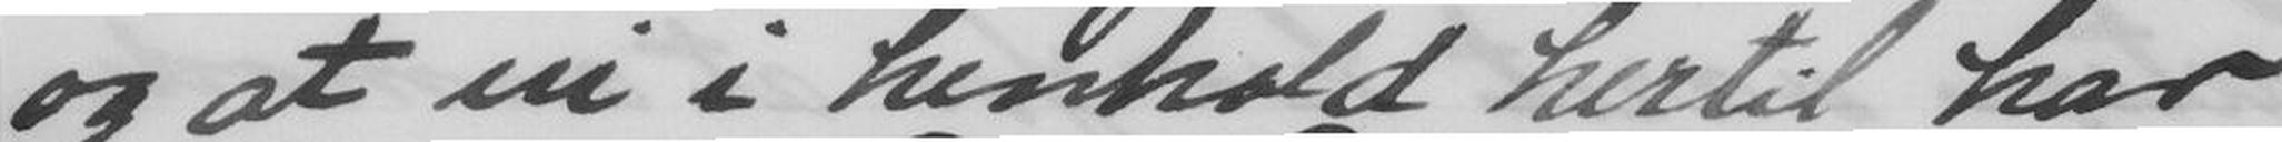og at vi i henhold hertil har
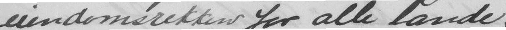eiendomsretten for alle lande,
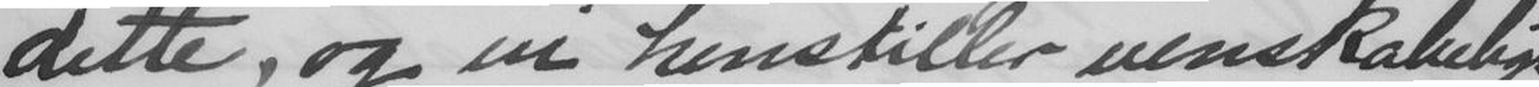dette, og vi henstiller venskabelig
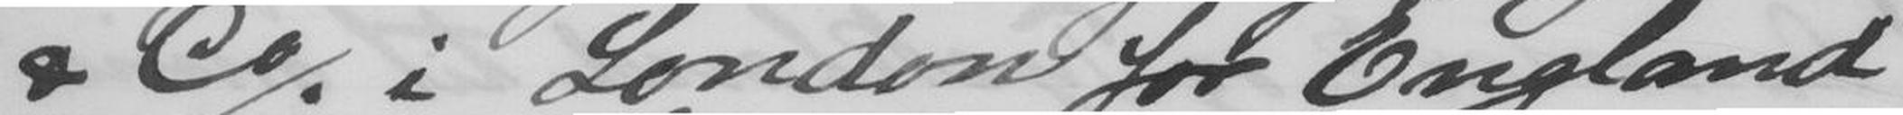& Co i London for England
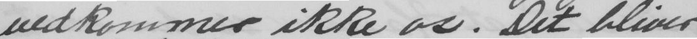vedkommer ikke os. Det bliver
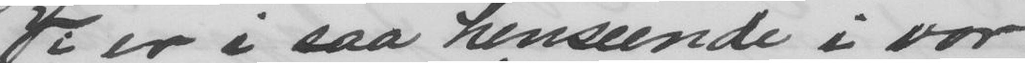Vi er i saa henseende i vor
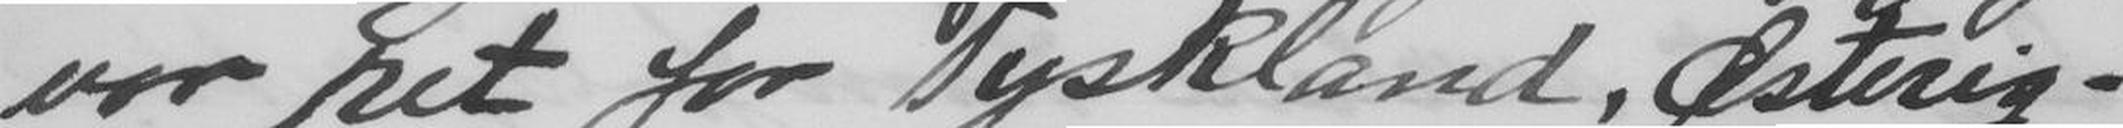vor ret for Tyskland, Østerig-
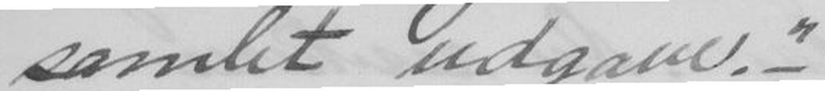samlet udgave."
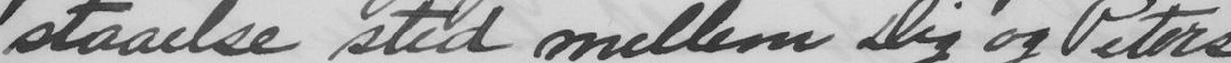staaelse sted mellem Dig og Peters
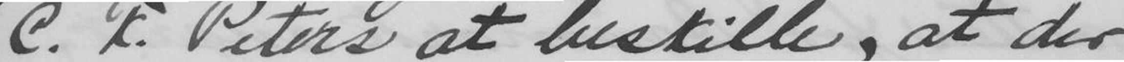C. F. Peters at bestille, at der
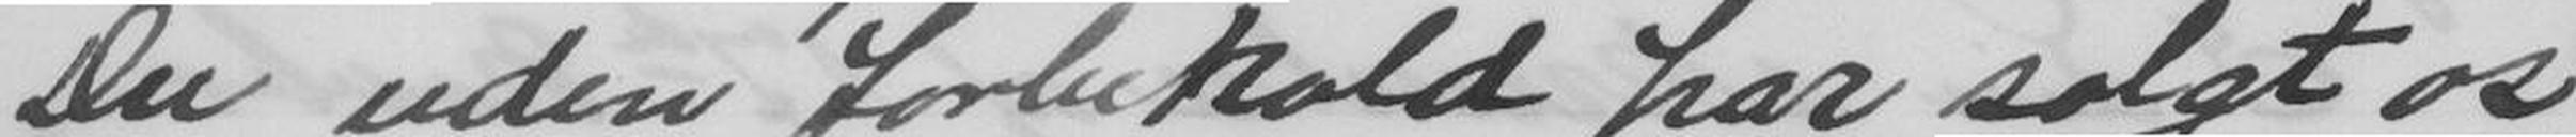Du uden forbehold har solgt os
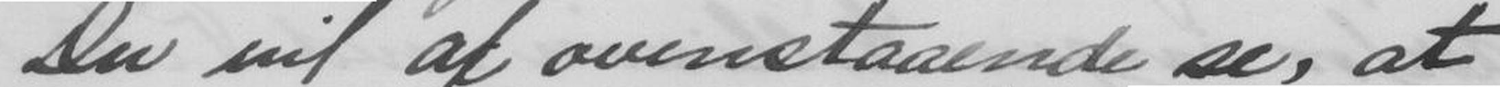Du vil af ovenstaaende se, at
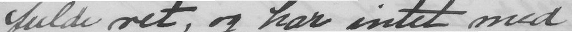fulde ret, og har intet med
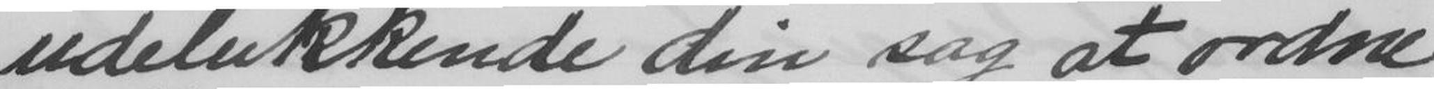udelukkende din sag at ordne
